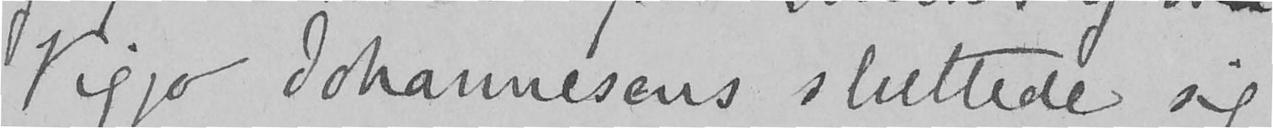Viggo Johannesens sluttede sig
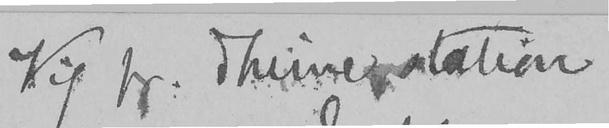Vig pr. Thime station
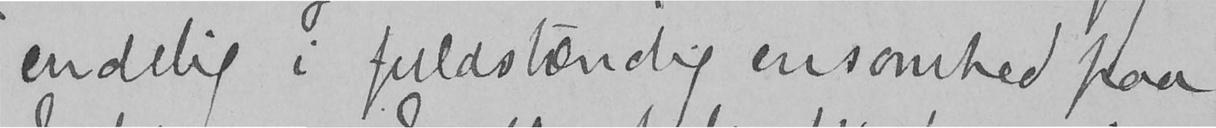endelig i fuldstændig ensomhed paa
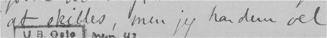at skilles, men jeg har dem vel
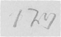179
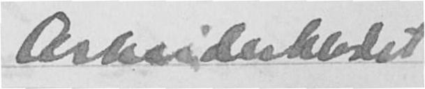Askerbladet
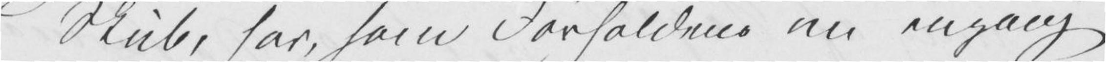Skub, har, som Forholdene nu engang
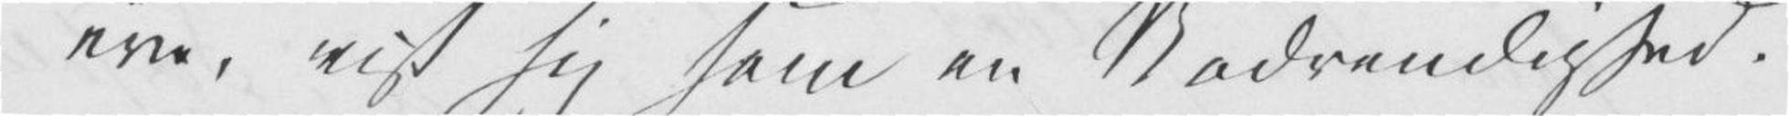ere, vist sig som en Nødvendighed.
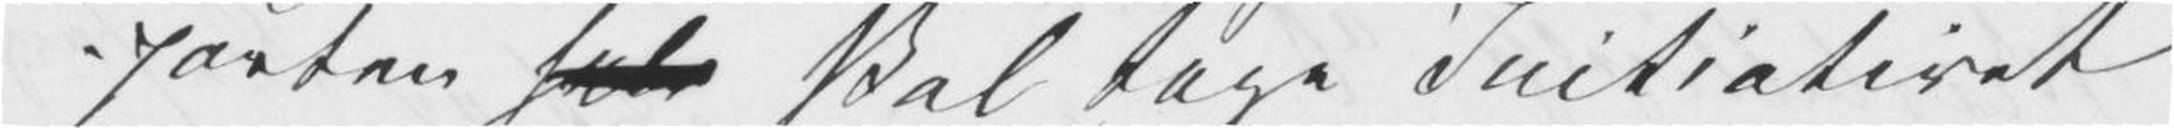parten selv skal tage Initiativet
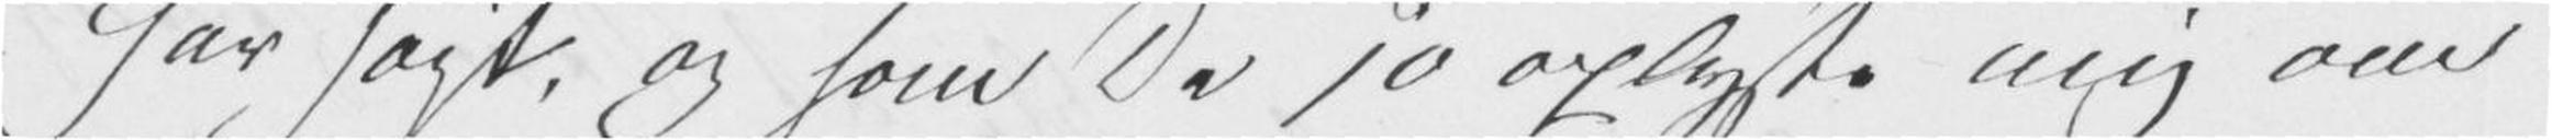har søgt, og som De jo oplyste mig om
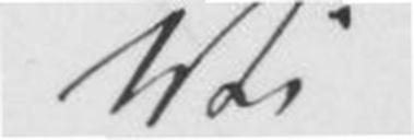Vi
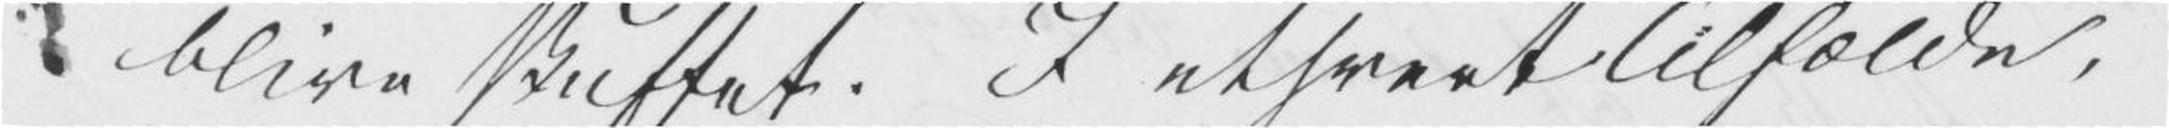blive skuffet. I ethvert Tilfælde,
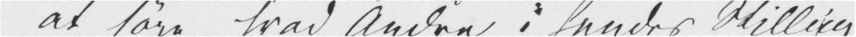at søge, hvad Andre i hendes Stilling
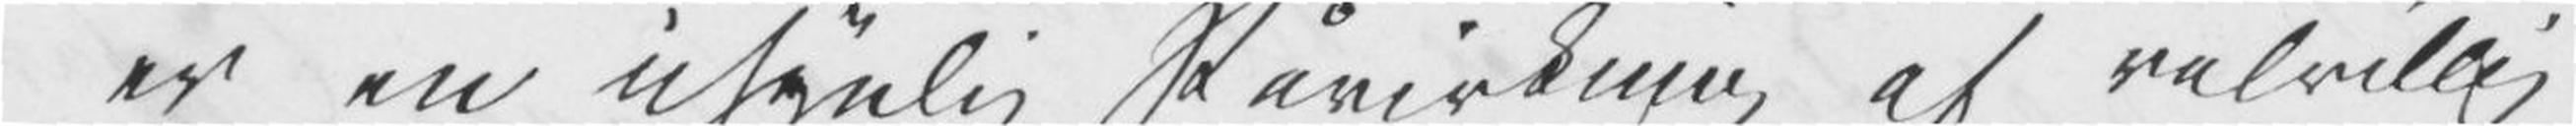er en usynlig Påvirkning af velvillig
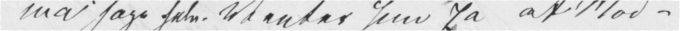må søge selv. Venter hun på at Mod¬
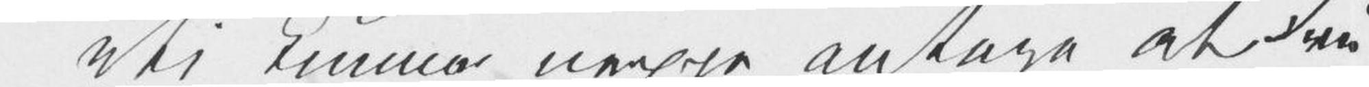Vi kunne neppe antage at Fru
